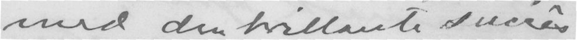med den brilliante sucsess
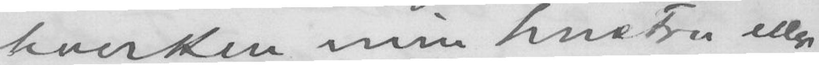hverken min hustru eller
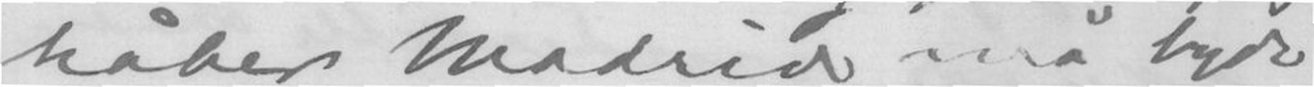håber Madrid må byde
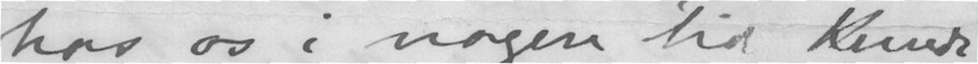hos os i nogen Tid, Kunde
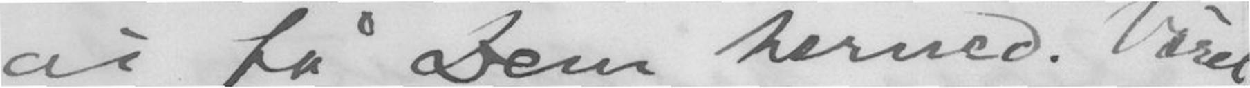at få Dem herned. Værel-
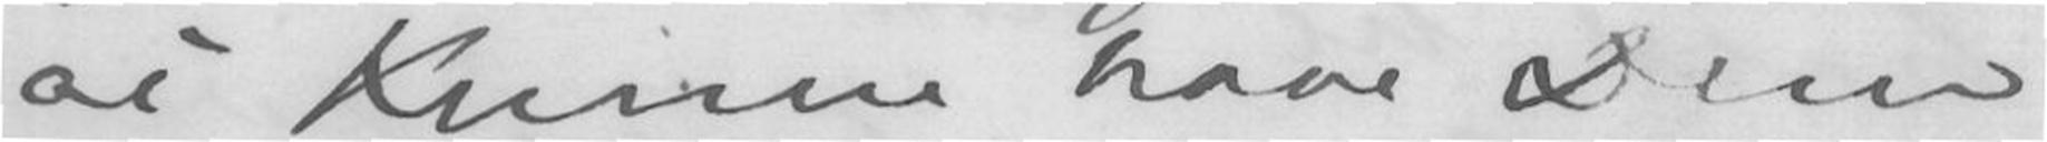at Kunne have Dem
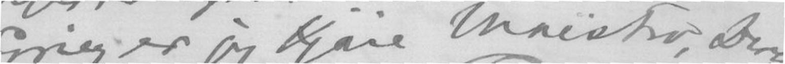Grieg er jeg Kjære Maëstro, Deres
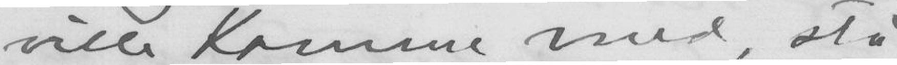ville Komme med, stå
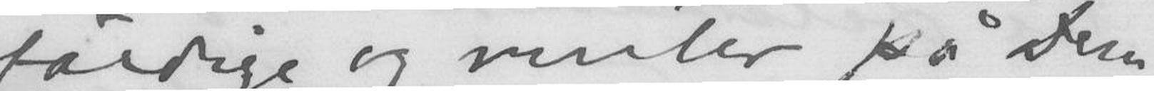færdige og venter på Dem
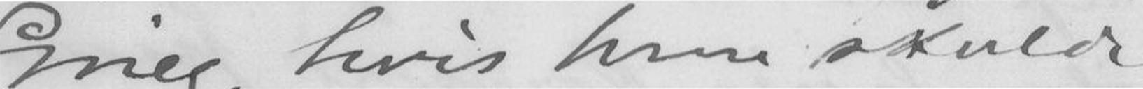Grieg, hvis hun skulde
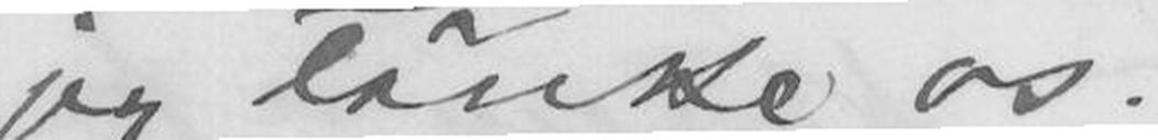jeg tænke os.
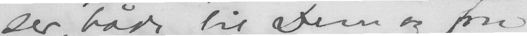ser både til Dem og fru
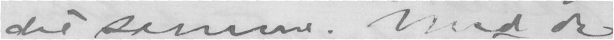det samme. Med de
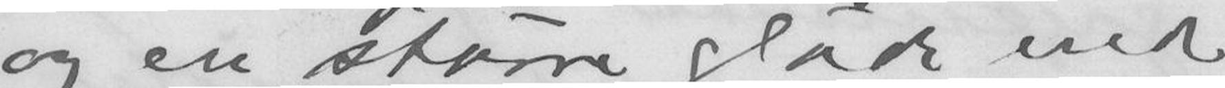og en større glæde end
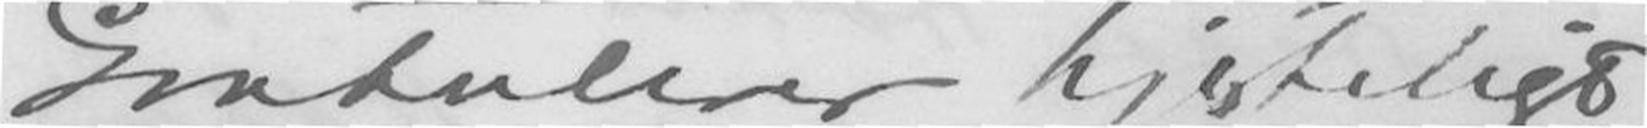Gratulerer hjerteligst
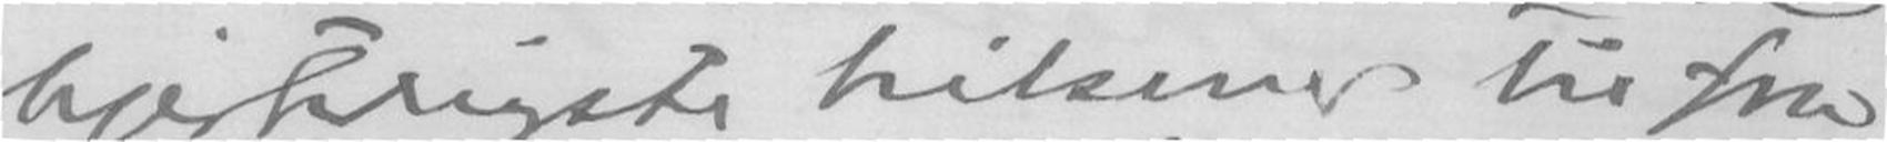hjerteligste hilsener til fru
